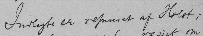Indlagte er refuseret af Holst;
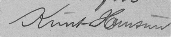Knut Hamsun.
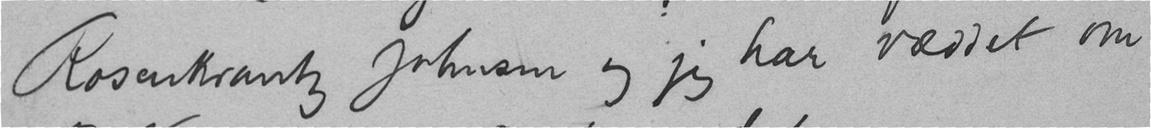Rosenkrantz Johnsen og jeg har væddet om
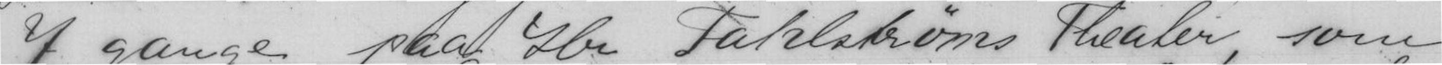7 gange paa Hr Tahlstrøms Theater, som
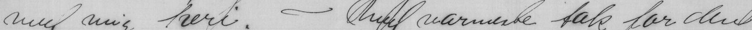med mig heri. - Med varmeste tak for den
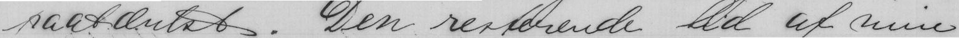paatænkt. Den resterende tid af min
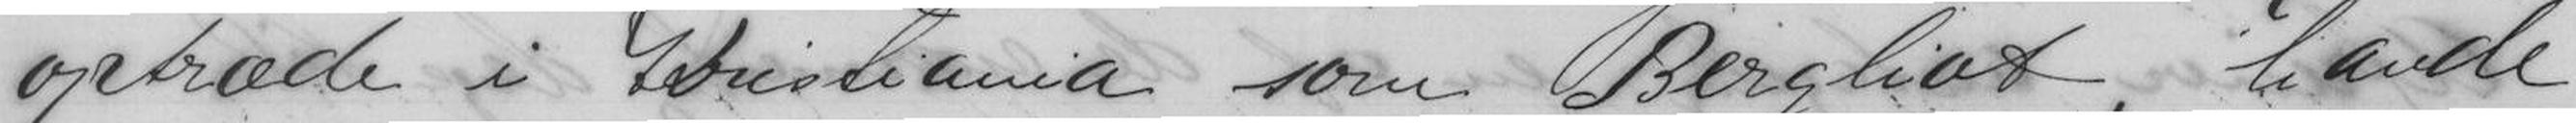optræde i Kristiania som Bergliot, havde
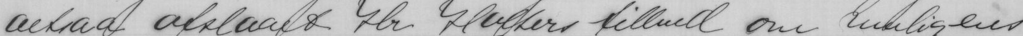altsaa afslaaet Hr Hytters tilbud om muligens
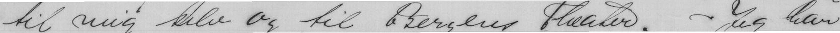til mig selv og til Bergens Theater. Jeg har
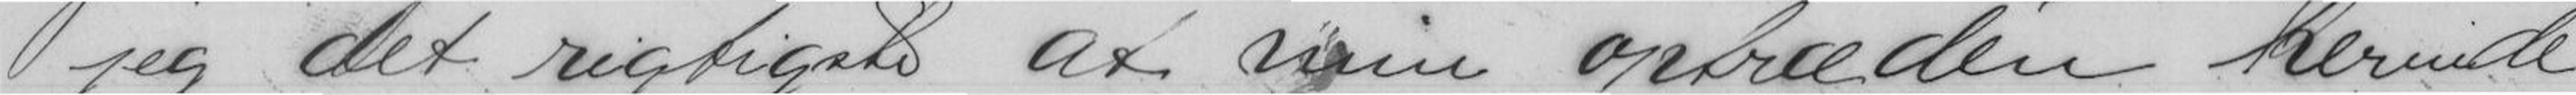jeg det rigtigste at min optræden herinde
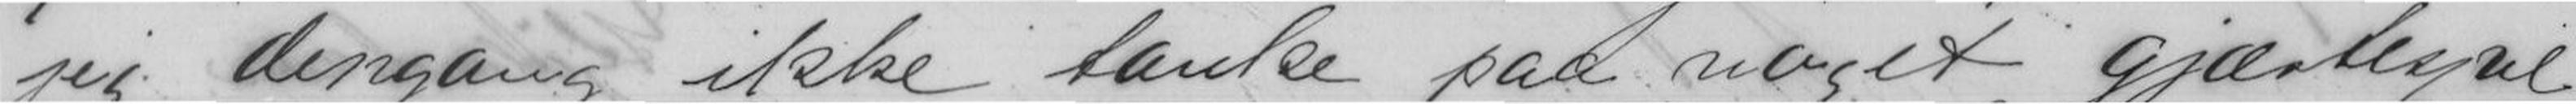jeg dengang ikke tanke paa noget gjæstespel
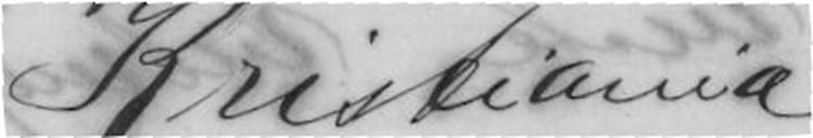Kristiania
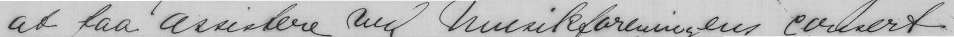at faa assistere ved Musikforeningens consert
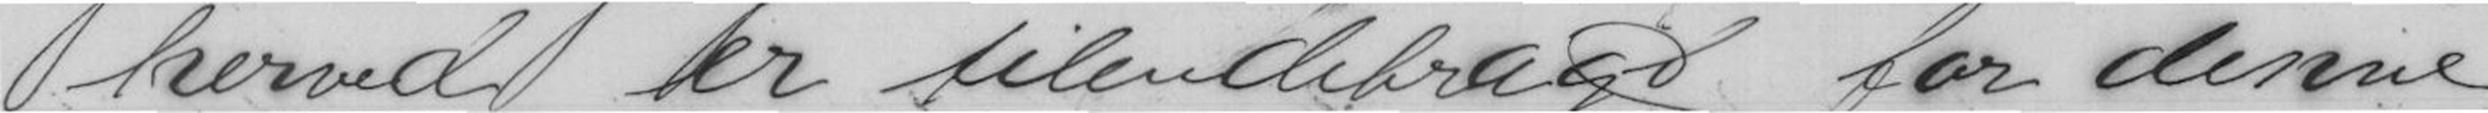herved er tilendebragt for denne
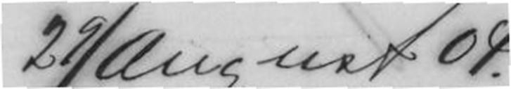29/August 04
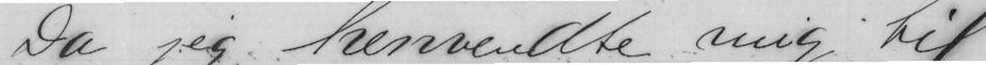Da jeg henvendte mig til
